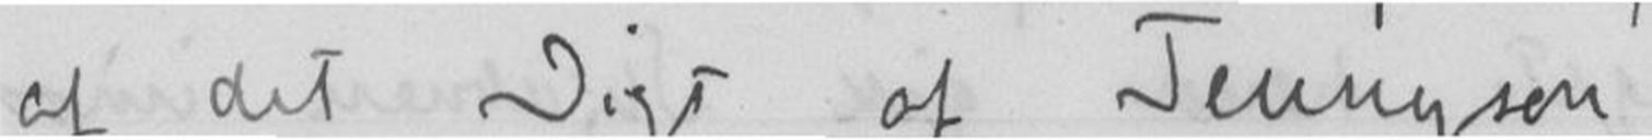af det Digt Tennyson,
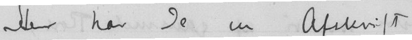Her har De en Afskrift
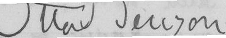Ottar Benzon
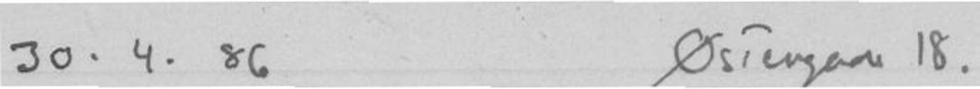30.4.86Østergade 18.
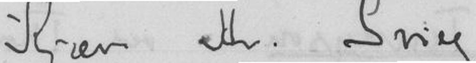Kjære Hr. Grieg!
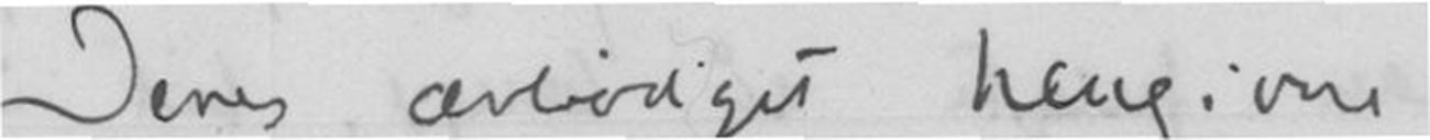Deres ærbødigst hengivne
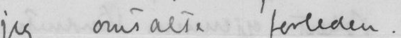jeg omtalte forleden.
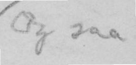Og saa
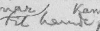til hende
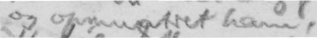og opmuntret ham,
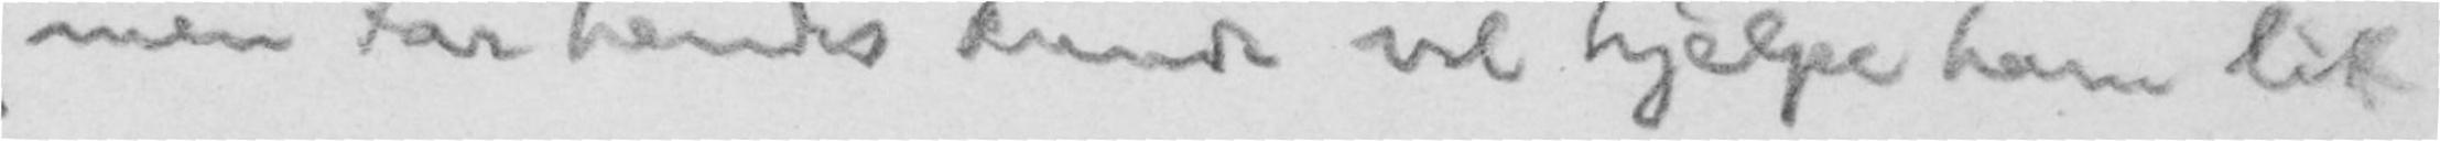men Far hendes kunde vel hjelpe ham litt
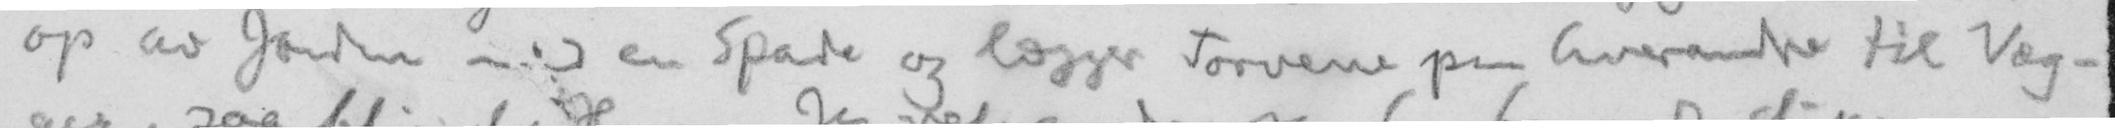op av Jorden med en Spade og lægger Torvene paa hverandre til Væg-
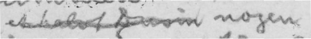et halvt Dusin nogen
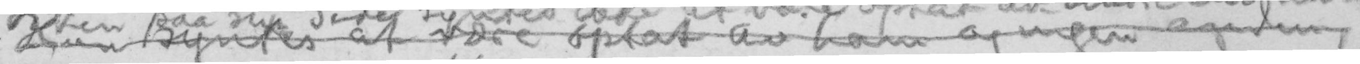Hun syntes at være optat av ham og ingen anden
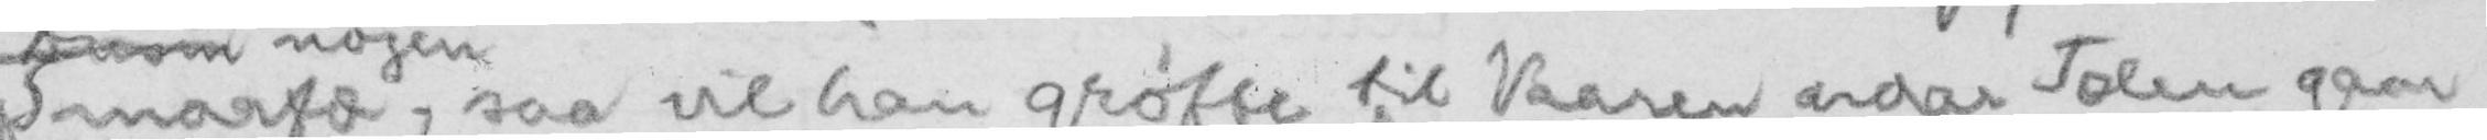Smaafæ, saa vil han grøfte til Vaaren naar Tælen gaar
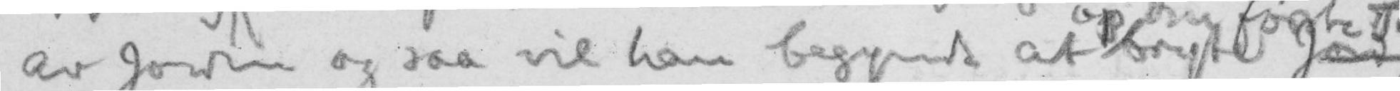av Jorden og saa vil han begynde at bryte Jord
